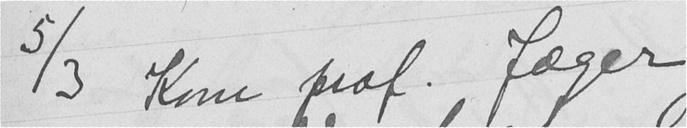5/3 Kom prof. Jæger
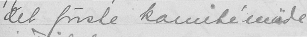det første komitémøde
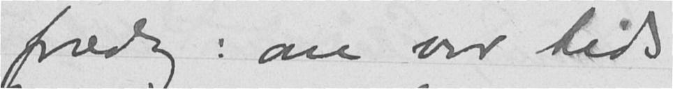foredrag: om vor tids
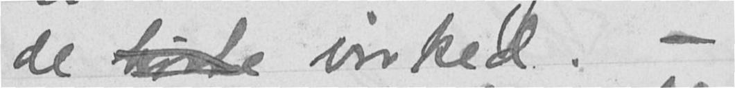de hørte virked. -
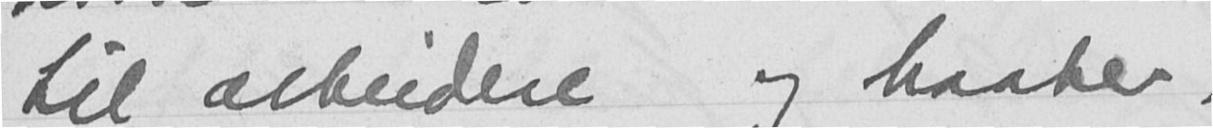til arbeidere og haaber,
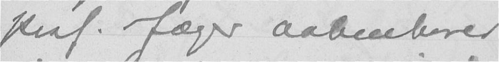prof. Jæger aabenbarer
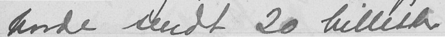havde sendt 20 billetter
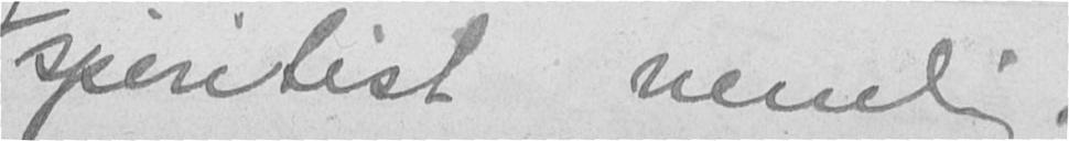spiritist nemlig.
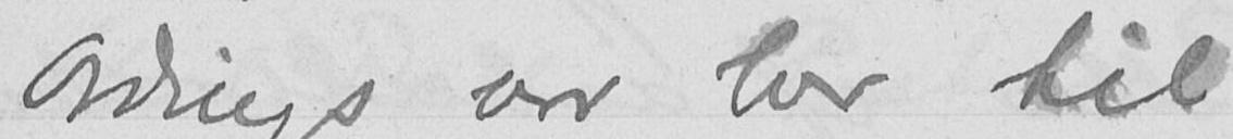Ordings var her til
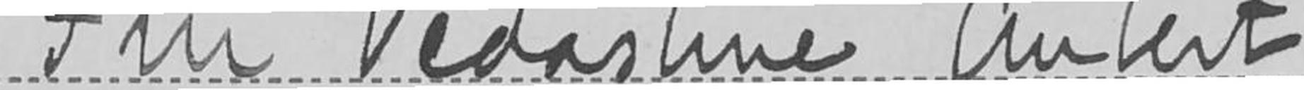Fru Vedastine Aubert
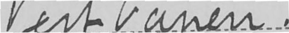Vestbanen.
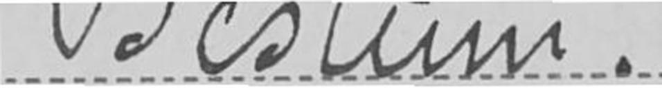Bestum.
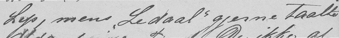Lys, mens "Ledaal" gjerne taalte
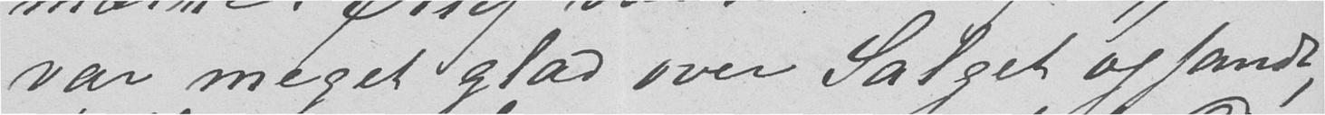var meget glad over Salget og fandt,
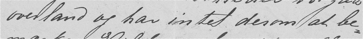over land og har intet derom at be-
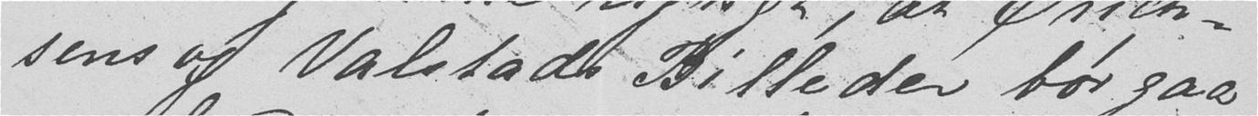sens og Valstads Billeder bør gaa
